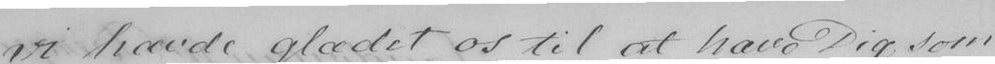vi havde glædet os til at have Dig som
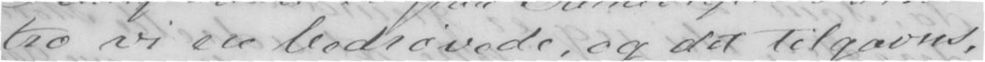tro vi ere bedrøvede, og det tilgavns,
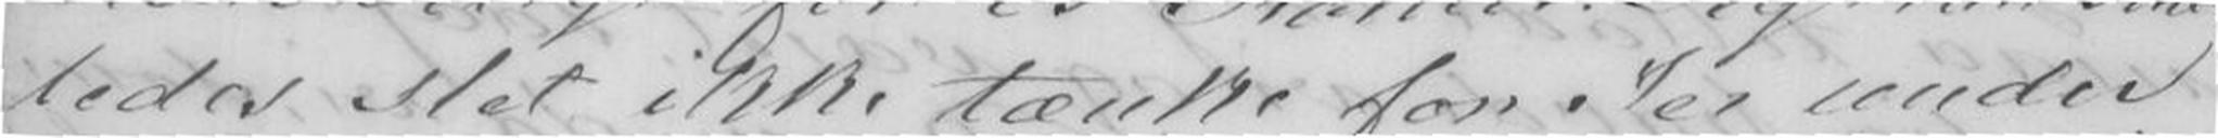ledes slet ikke tænke for Jer under
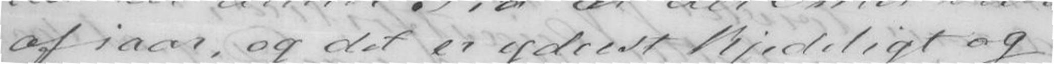af iaar, og det er yderst kjædeligt og
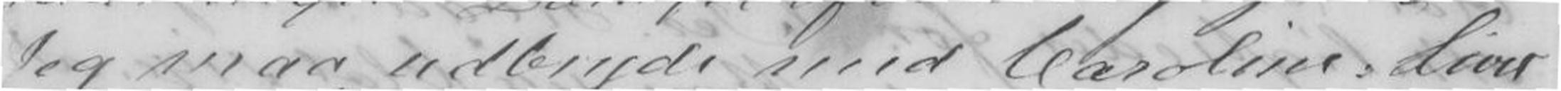Jeg maa udbryde med Caroline: "Livet
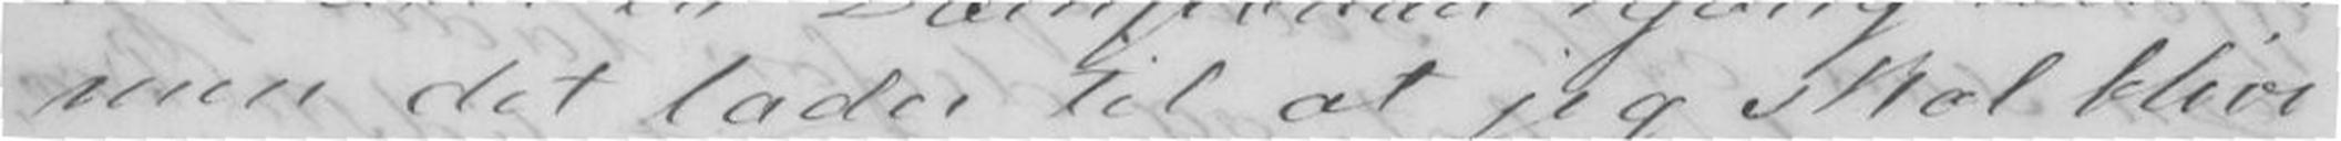men det lader til at jeg skal blive
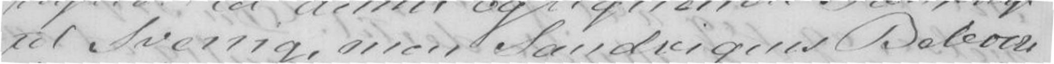til Sverrig, men Sandvigens Beboere
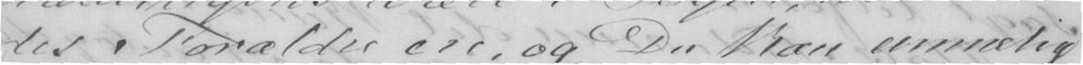des Forældre ere, og Du kan umuelig
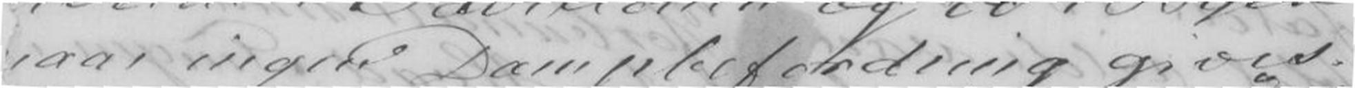aar ingen Dampbefordring gives.
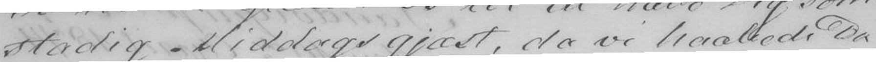stadig Middaggjæst, da vi haabede Du
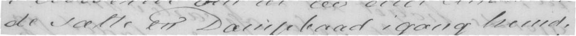de sætte en Dampbaad igang herud,
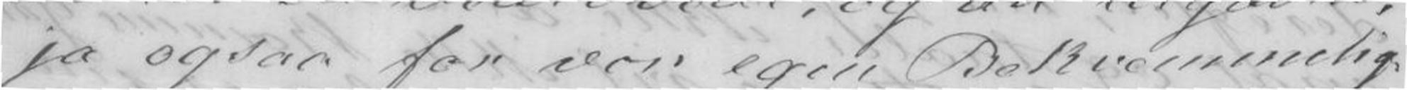ja ogsaa for vor egen Bekvemmelig-
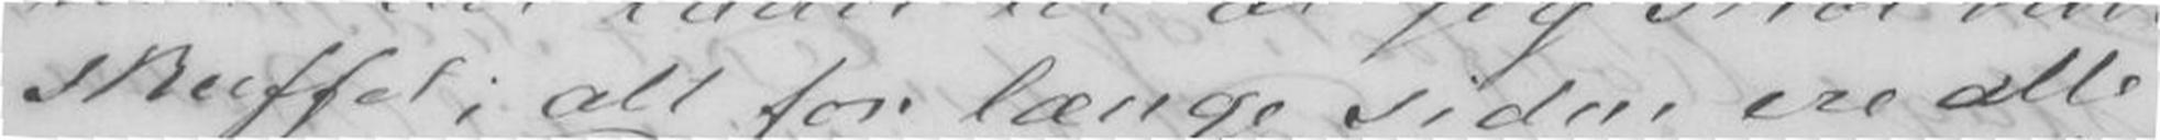skuffet; alt for længe siden ere alle
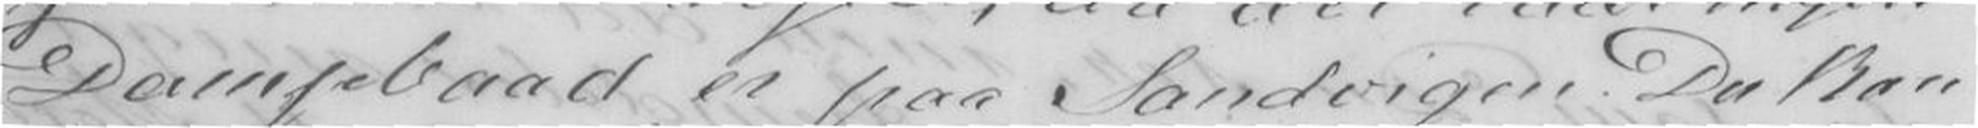Dampbaad er paa Sandvigen. Du kan
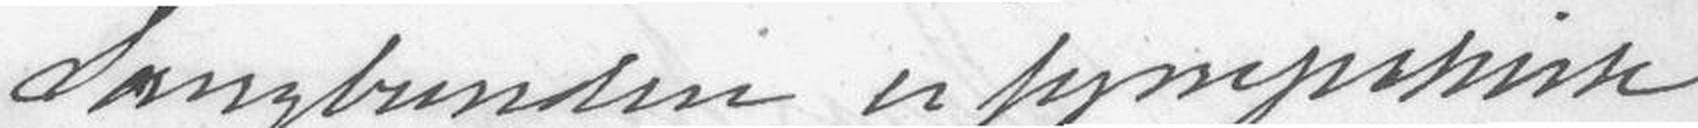Sangbunden er sympatisk,
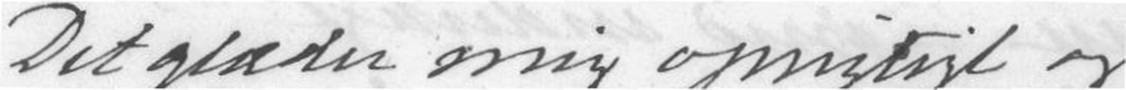Det glæder mig oprigtigt og
